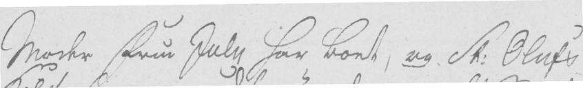Moder Frue July har boet, og St. Olufs
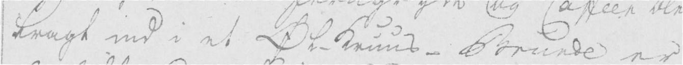bragt ind i et Øl-Kruus - Brude er
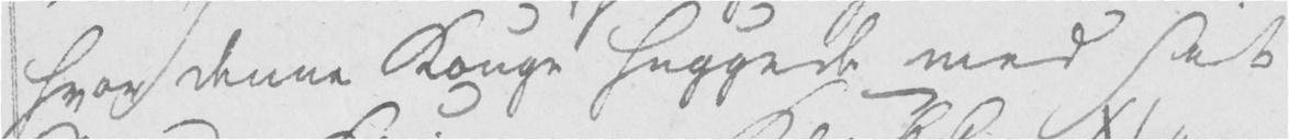hvor denne Konge huggede med sit
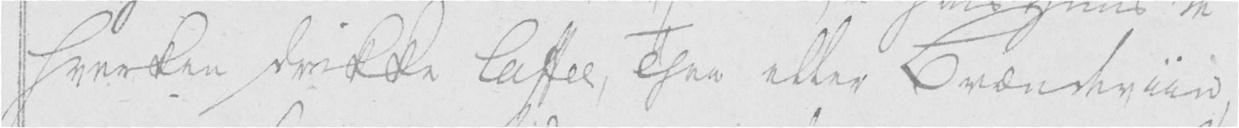hverken drikke Caffee, Thee eller Brændeviin,
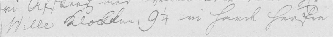Wille Klokken 9 1/4 vi havde herfra
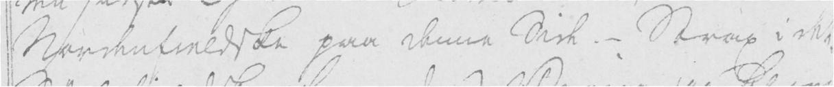Nordenfieldske paa denne Side. Strax i det
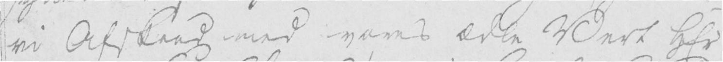vi Afskeed med vores ædle Vert Hr
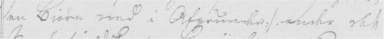en Biørn ned i Afgrunden :/ ender det
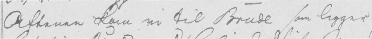Aftenen kom vi til Brude som ligger
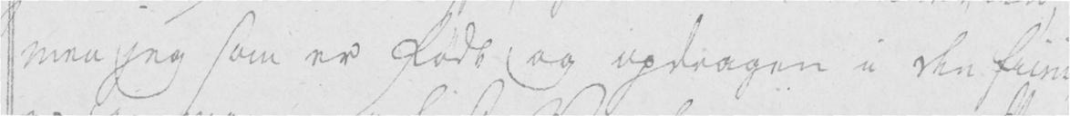men jeg som er født og opdragen i den fine-
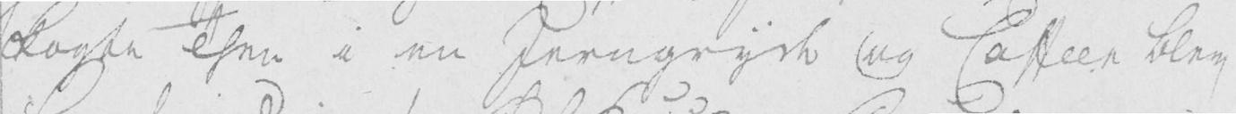kogte Thee i en Jerngryde og Caffeen blev
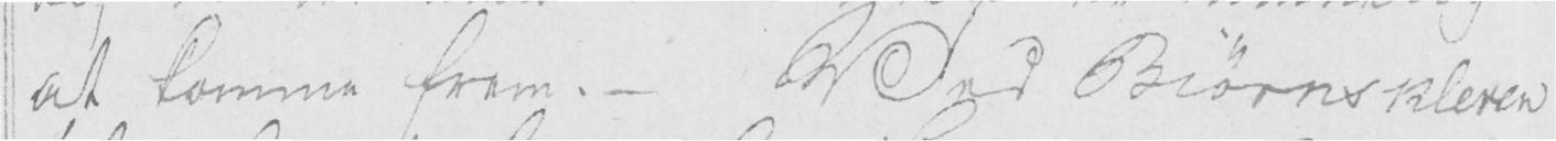at komme frem. - Ved Biørnskleven
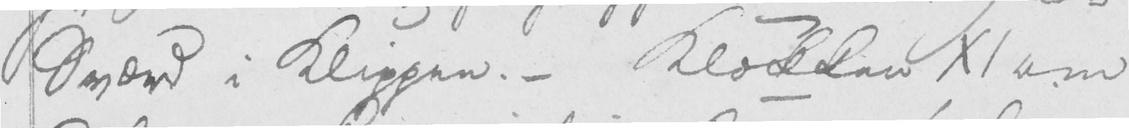Sværd i Klippen. - Klokken XI om
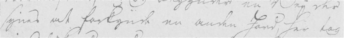synes at forkynde en anden Jord, her tog
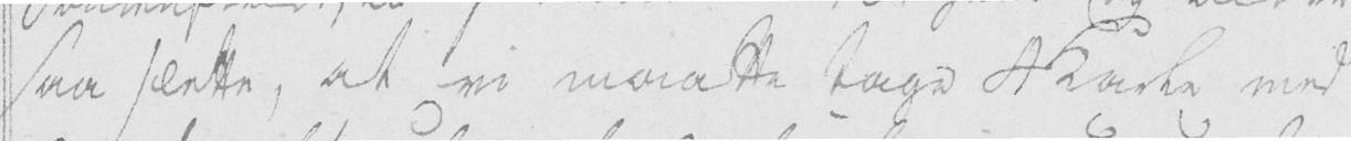saa slette, at vi maatte tage 4 Karle med
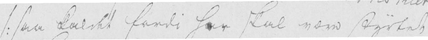/: saa kaldet fordi her skal være styrtet
